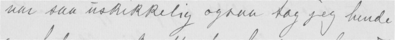var saa uskikkelig ogsaa tog jeg hende
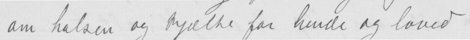om halsen og kjælte for hende og loved
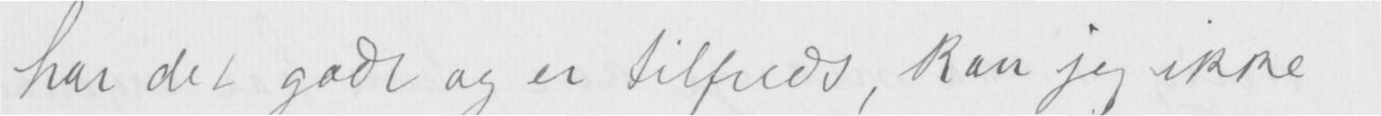har det godt og er tilfreds, kan jeg ikke
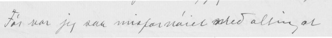Før var jeg saa misfornøiet med alting at
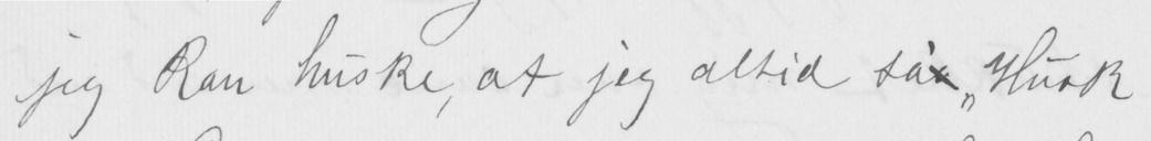jeg kan huske, at jeg altid saa "Husk
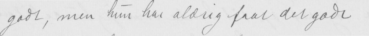godt, men hun har aldrig faat det godt
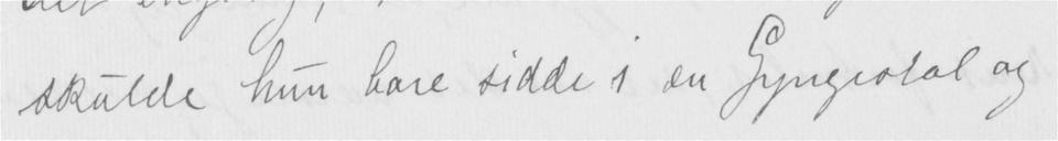skulde hun bare sidde i en Gyngestol og
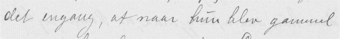det engang, at naar hun blev gammel
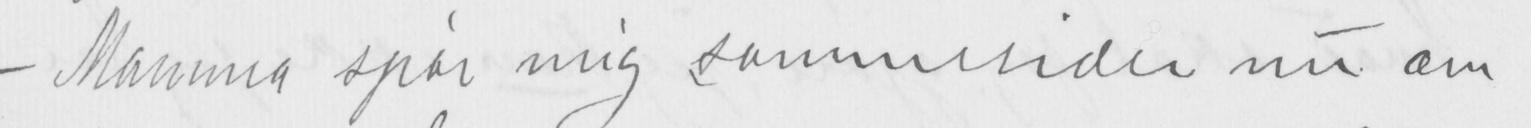– Mamma spør mig sommetider nu om
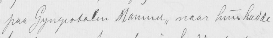paa Gyngestolen Mamma" naar hun hadde
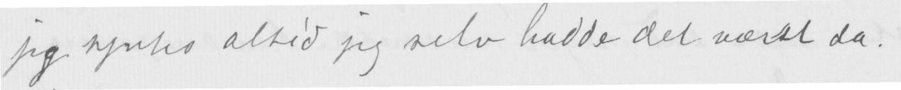jeg syntes altid jeg selv hadde det værst da.
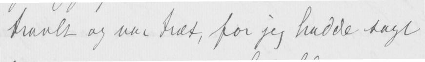travlt og var træt, for jeg hadde sagt
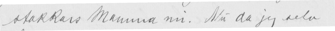stakkars Mamma mi. Nu da jeg selv
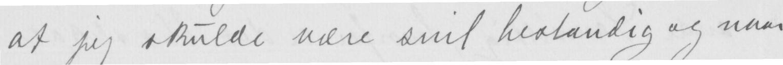at jeg skulde være snil bestandig og naar
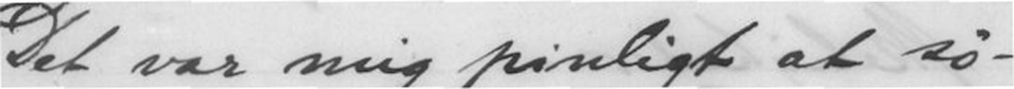Det var mig pinligt at sø-
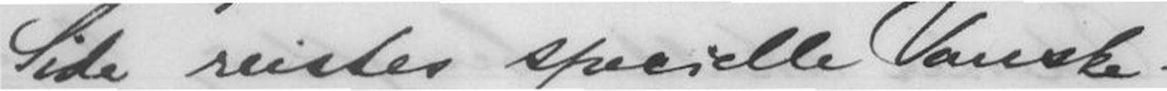Side reiste specielle Vanske-
